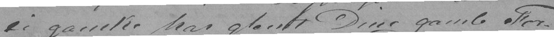ei ganske har glemt Dine gamle For-
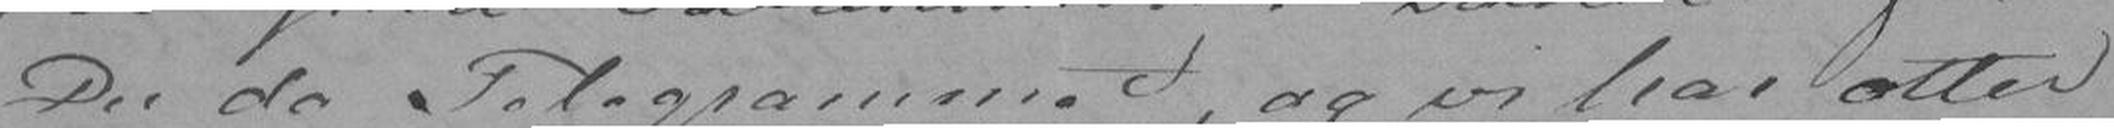Du da Telegrammet, og vi har atter
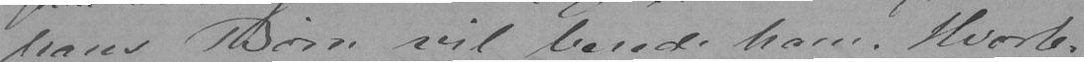hans Børn vil berede ham. Hvorle-
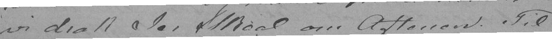vi drak Jer Skaal om Aftenen. Til
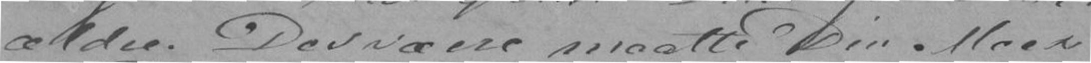ældre. Desværre maatte Din Moer
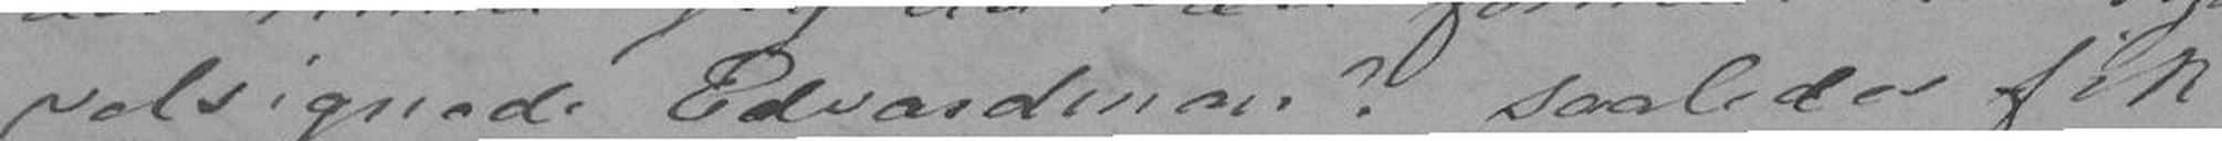velsignede Edvardman? saaledes fik
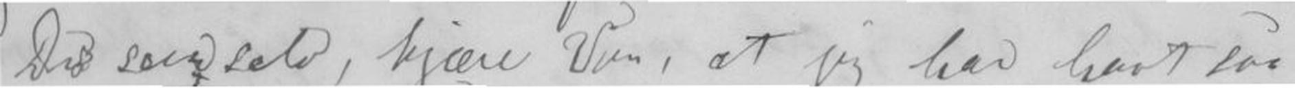Du ser selv, kjære Ven, at jeg har havt saa
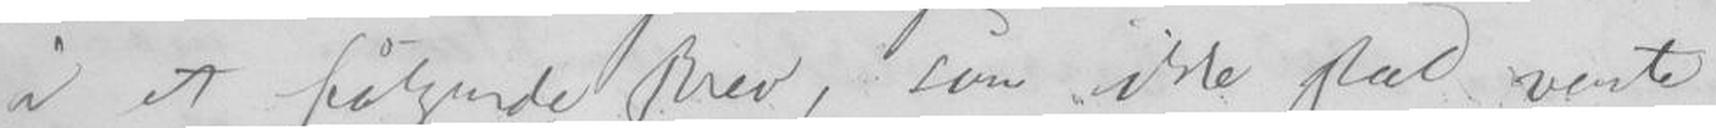i et følgende Brev, som ikke skal vente
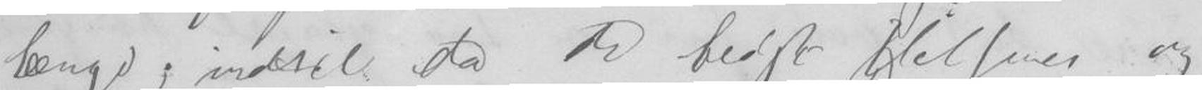længe, indtil da de bedste Hilsner og
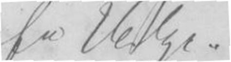fra Helga.
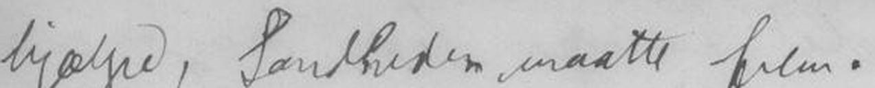hjælpe, Sandheden maatte frem.
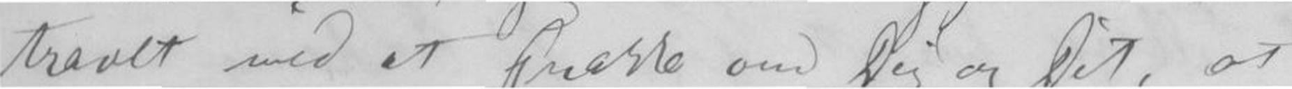travelt med at snakke om Dig og Dit, at
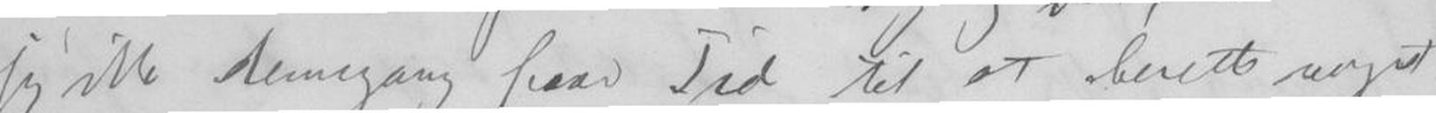jeg ikke dennegang faar Tid til at berette noget
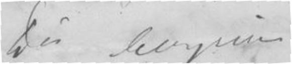Din hengivne
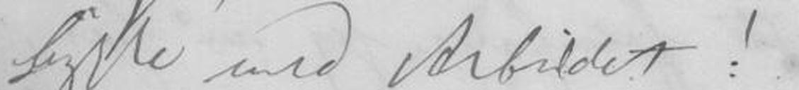lykke med Arbeidet!
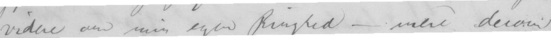videre om min egen Ringhed - mere derom
